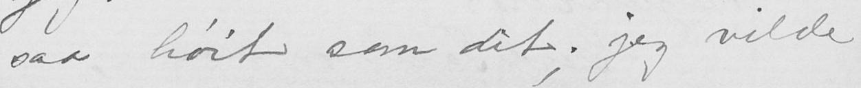saa høit som dit; jeg vilde
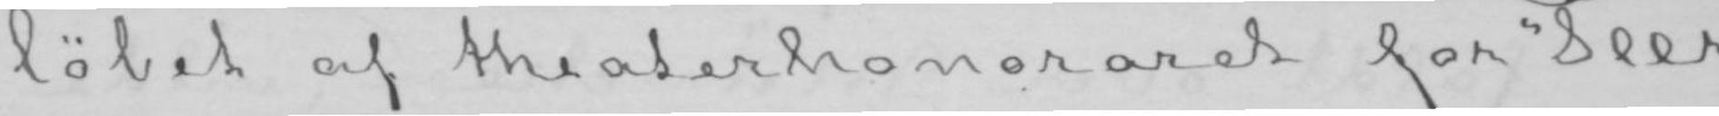løbet af theaterhonoraret for «Peer
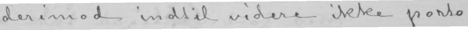derimod indtil videre ikke porto
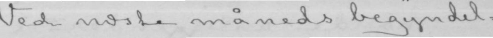Ved næste måneds begyndel-
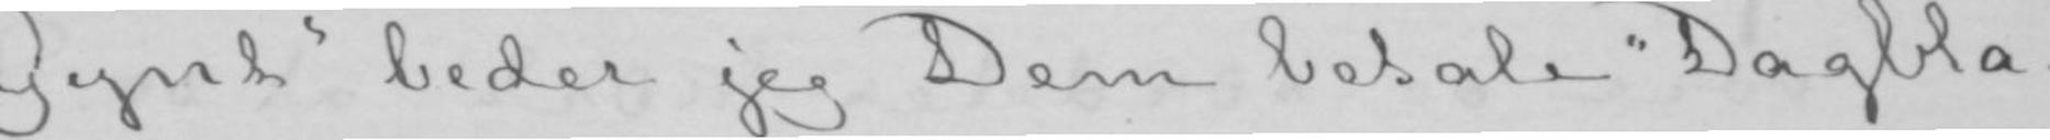Gynt» beder jeg Dem betale «Dagbla-
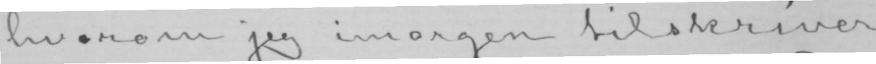hvorom jeg imorgen tilskriver
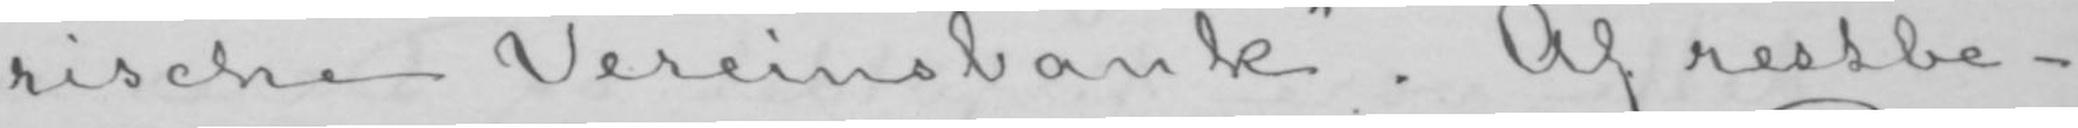rische Vereinsbank». Af restbe-
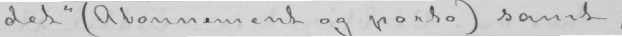det» (Abonnement og porto) samt,
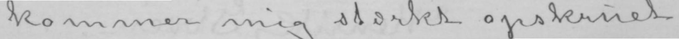kommer mig stærkt opskruet,
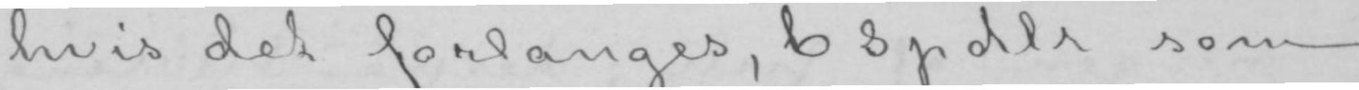hvis det forlanges, 6 spdlr som
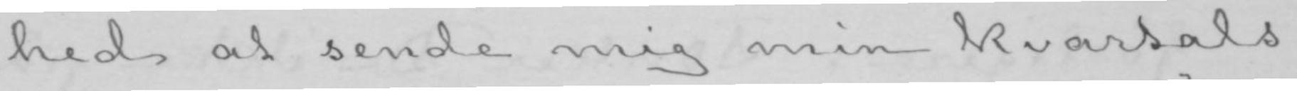hed at sende mig min kvartals-
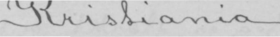Kristiania.
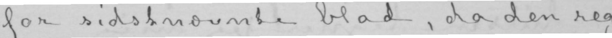for sidstnævnte blad, da den reg-
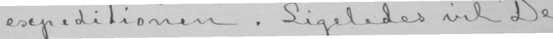expeditionen. Ligeledes vil De
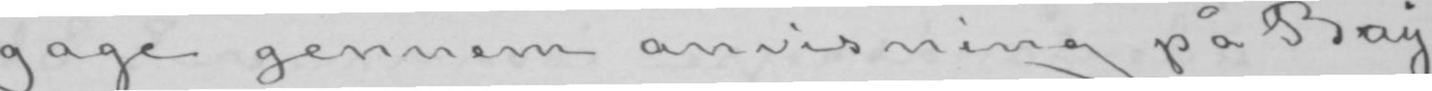gage gennem anvisning på «Bay-
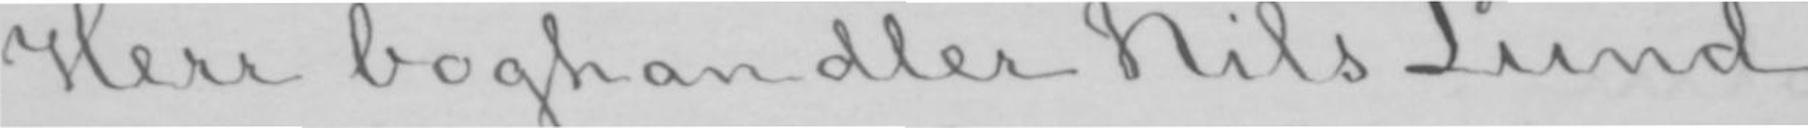Herr boghandler Nils Lund.
